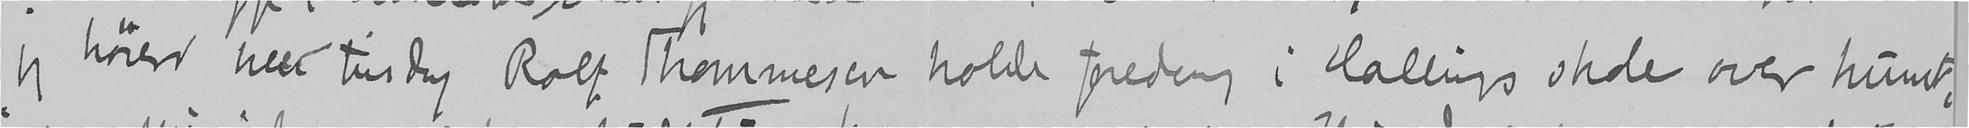jeg hører hver tirsdag Rolf Thommesen holde foredrag i Hallings skole over kunst,
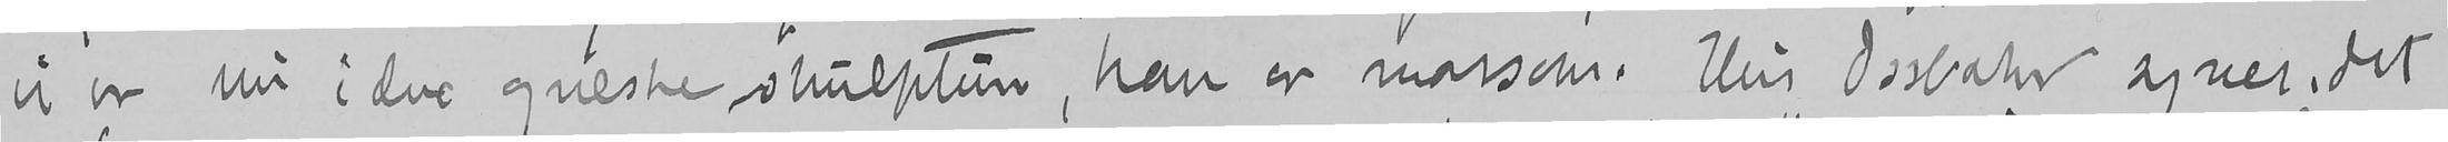vi er nu i den greske skulptur, han er morsom. Hvis Ossbahr synes, det
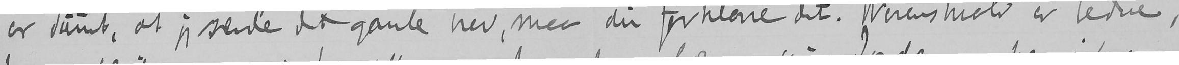er dumt, at jeg sende det gamle brev, maa du forklare det. Werenskiold er bedre,
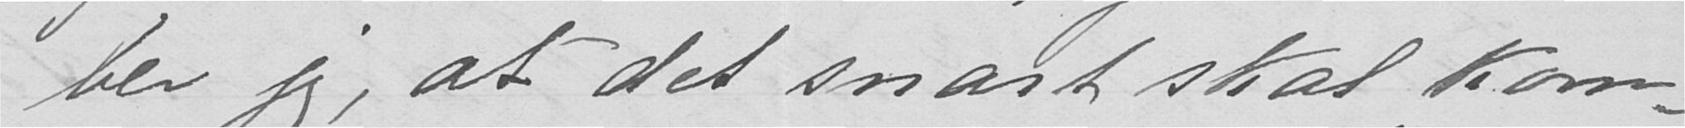ber jeg, at det snart skal kom-
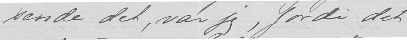sende det, var jeg, fordi det
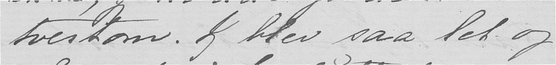tvertom. Jeg blev saa let og
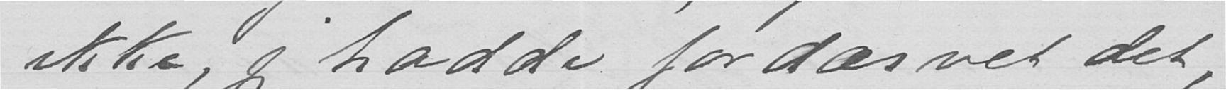ikke, jeg hadde fordærvet det,
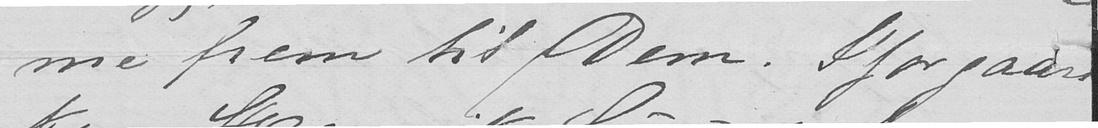me frem til Dem. I forgaars
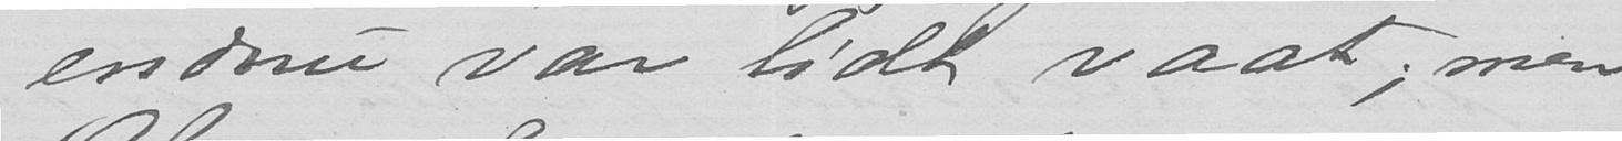endnu var lidt vaat; men
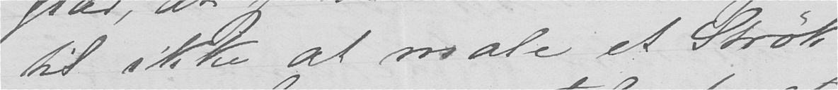til ikke at male et Strøk
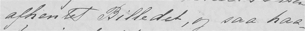afhentet Billedet, og saa haa-
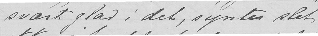svært glad i det, syntes slet
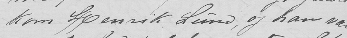kom Henrik Lund, og han var
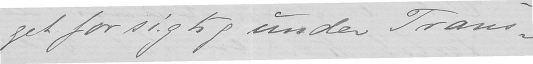get forsigtig under Trans-
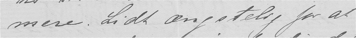mere. Lidt ængstelig for at
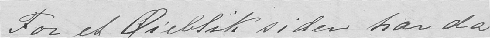For et Øieblik siden har da
